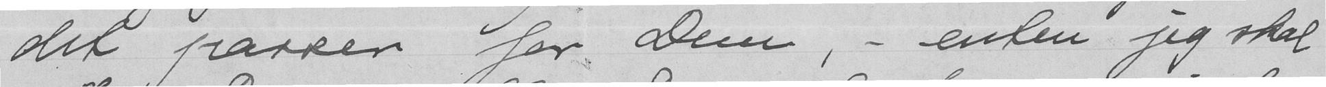det passer for Dem, - enten jeg skal
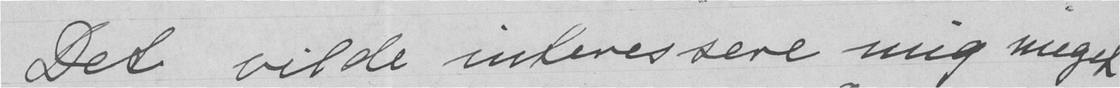Det vilde interessere mig meget
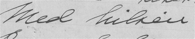Med hilsen
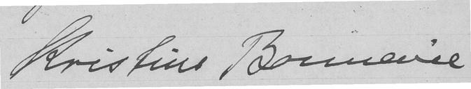Kristine Bonnevie
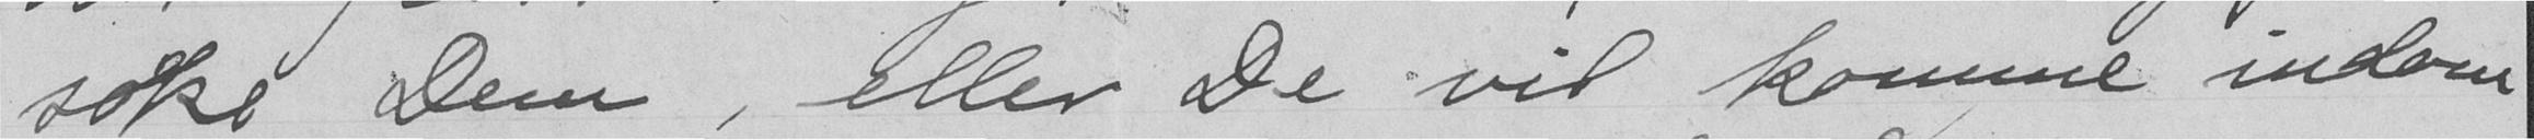søke Dem, eller De vil komme indom
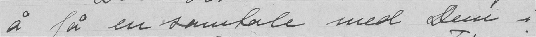å få en samtale med Dem i
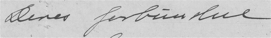Deres forbundne
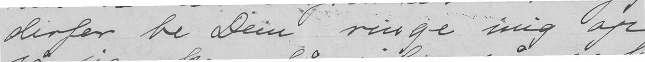derfor be Dem ringe mig op
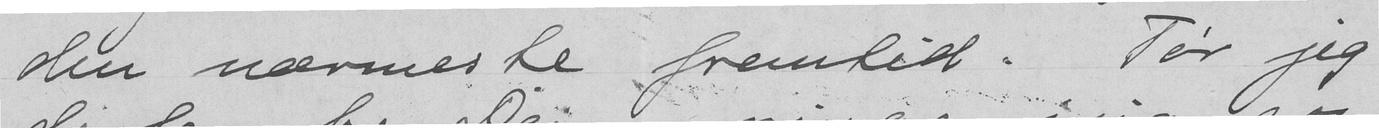den nærmeste fremtid. Tør jeg
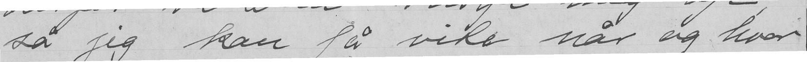så jeg kan få vite når og hvor
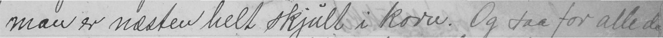man er næsten helt skjult i korn. Og saa for alle de
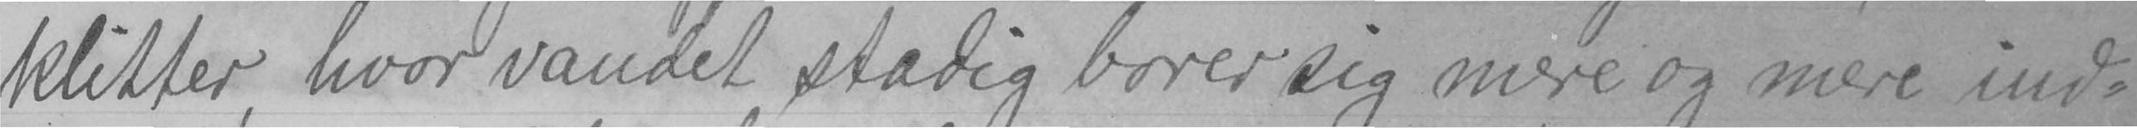klitter, hvor vandet stadig borer sig mere og mere ind-
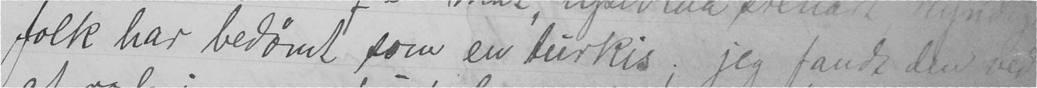folk har bedømt som en turkis; jeg fandt den ved
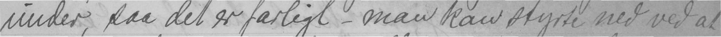under, saa det er farligt - man kan styrte ned ved at
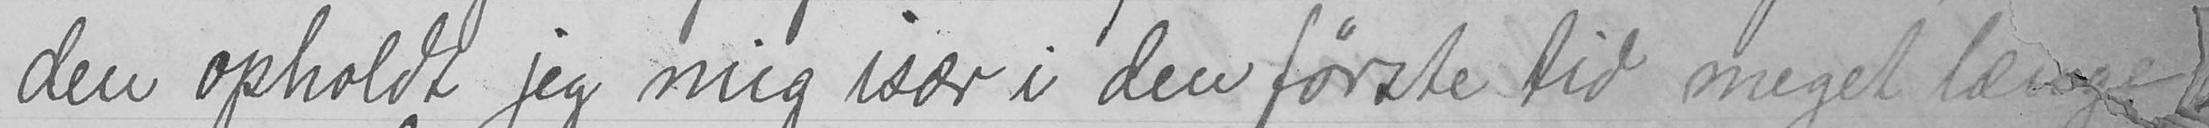den opholdt jeg mig især i den første tid meget længe
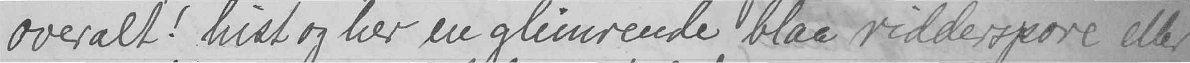overalt! hist og her en glimrende blaa ridderspore eller
